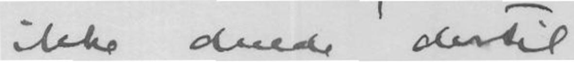ikke duede dertil.
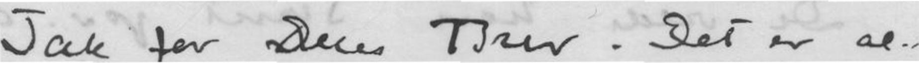Tak for Deres Brev. Det er al-
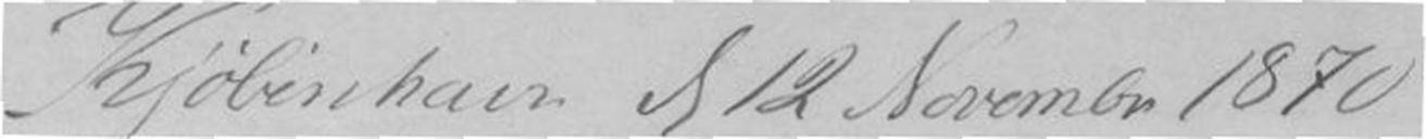Kjøbenhavn d 12 november 1870
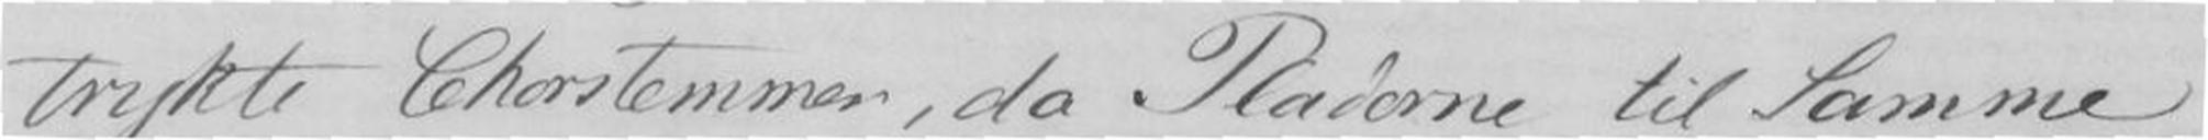trykte Chorstemmer, da Pladerne til Samme
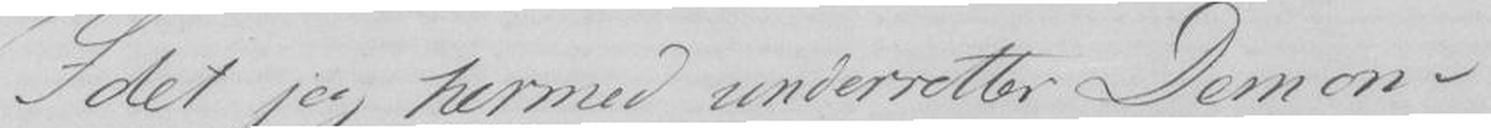Idet jeg hermed underretter Dem om
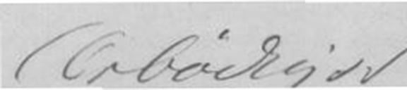Ærbødiged
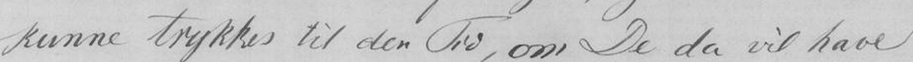kunne trykkes til den Tid, om De da vil have
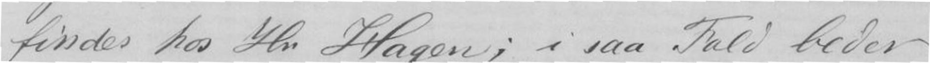findes hos Hr. Hagen; i saa fald beder
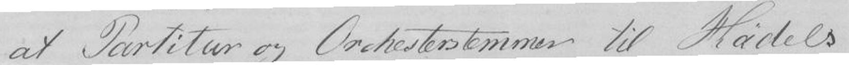at Partitur og Orchesterstemmer til Hädels
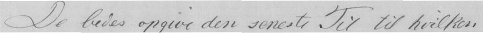De bedes opgive den seneste Tid til hvilken
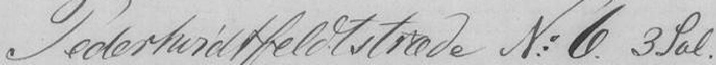Pederhvidtfeldtstræde N:6. 3Sal.
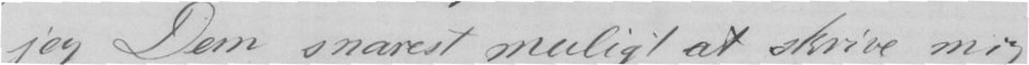jeg Dem snarest mulig at skrive mig
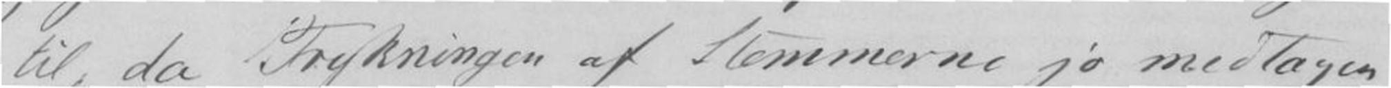til, da Trykningen af Stemmerne jo medtager
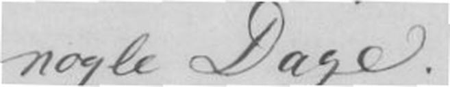nogle Dage.
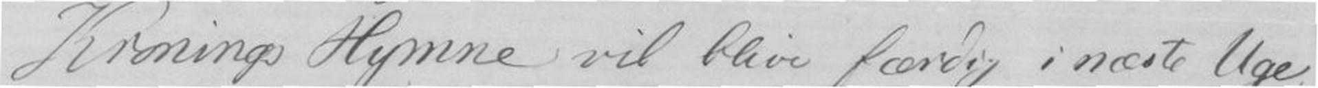Kroning Hymne vil blive færdig i næste Uge,
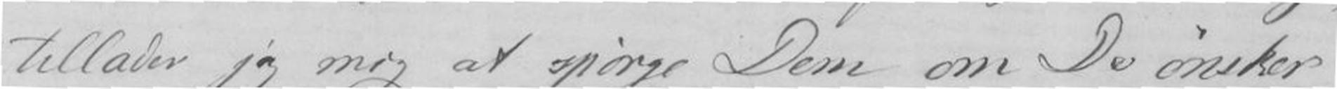tillader jeg mig at spørge Dem om De ønsker
